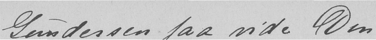Gundersen faa vide Din
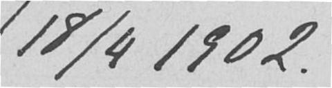18/4 1902.
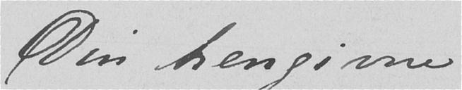Din hengivne
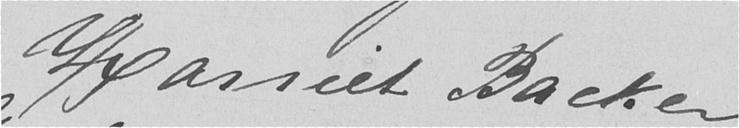Harriet Backer
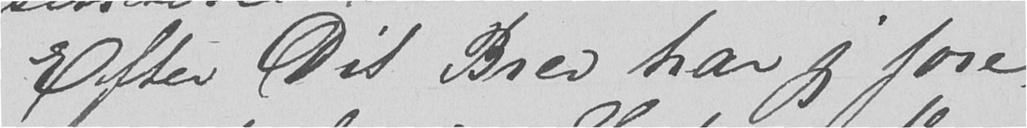Efter Dit Brev har jeg fore-
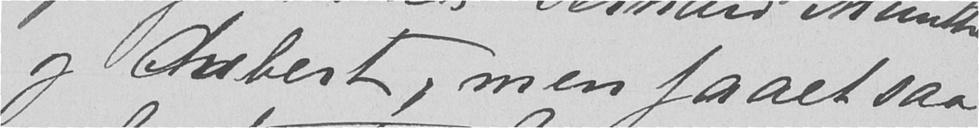og Aubert, men faaet saa
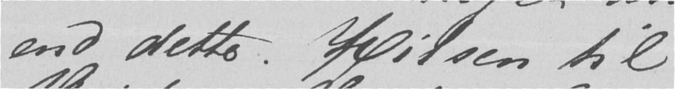end dette. Hilsen til
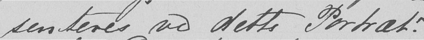senteres ved dette Portræt?
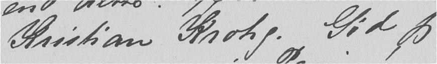Kristian Krohg. Gid jeg
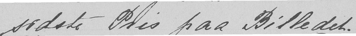sidste Pris paa Billedet.
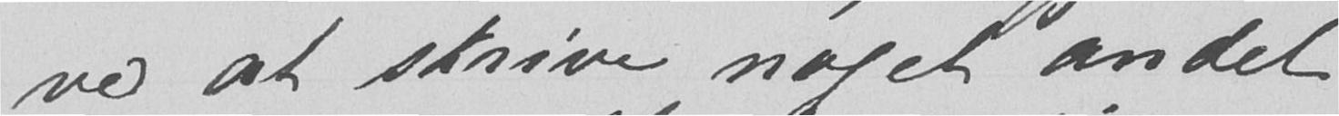ved at skrive noget andet
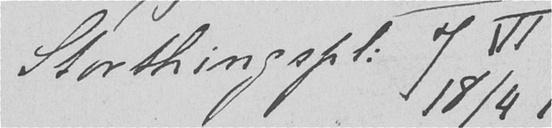Storthingspl. 7 VI
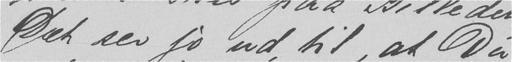Det ser jo ud til, at Du
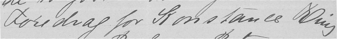Foredrag for Konstance Ring
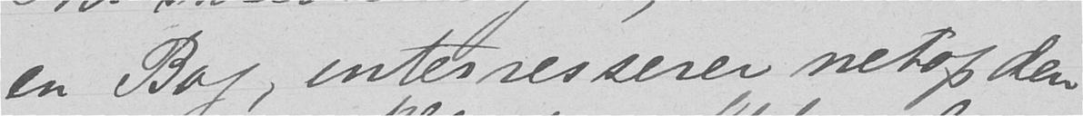en Bog interesserer netop den
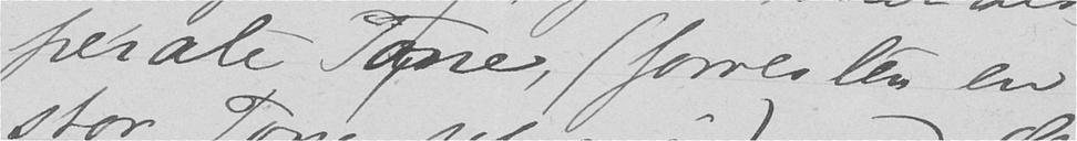perate Tone, (forresten en
